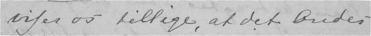viser os tillige, at det Ondes
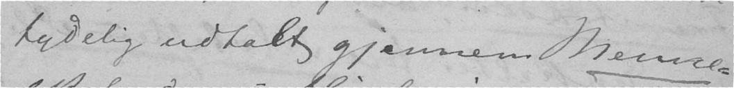tydelig udtalt gjennem Menne-
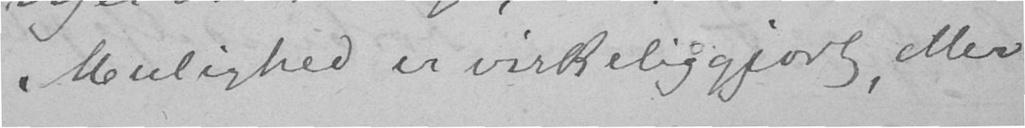Mulighed er virkeliggjort, eller
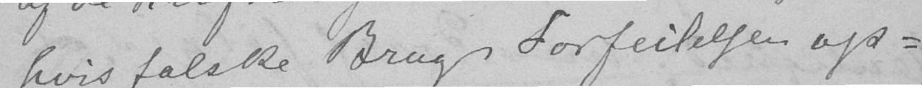hvis falske Brugs Forfeilelsen ops-
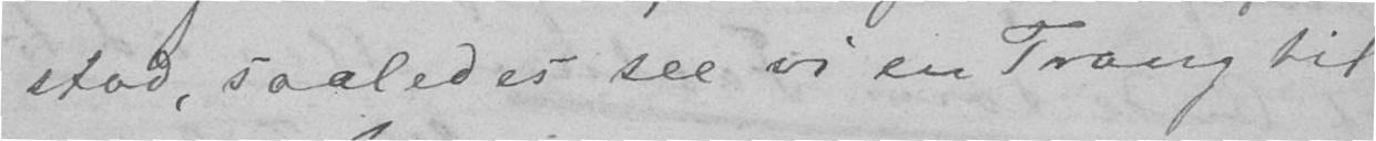stod, saaledes see vi en Trang til
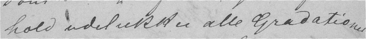hold udelukker alle Gradationer;
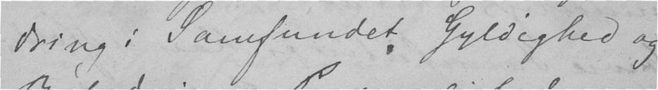dring i Samfundet Gyldighed og
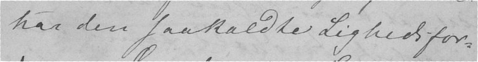har den saakaldte Lighedsfor-
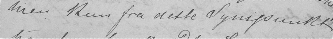men kun fra dette Synspunkt
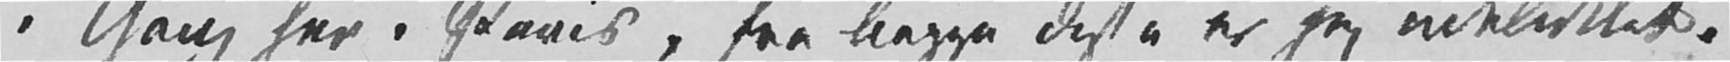i Gang her i Paris, fra begge disse er jeg udelukket.
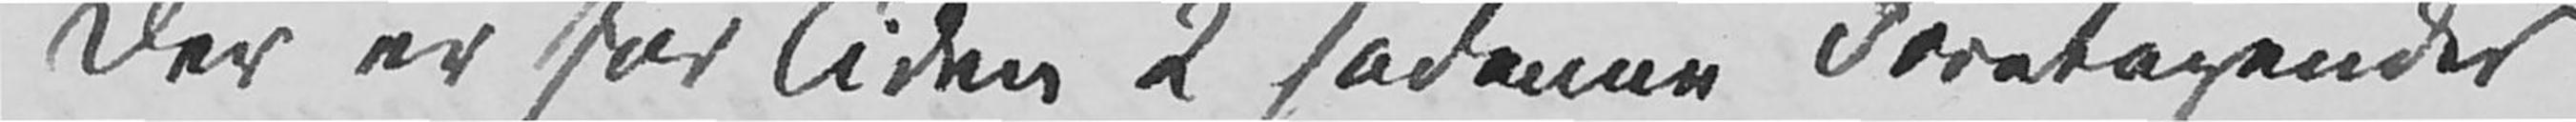Der er for Tiden 2 sådanne Foretagender
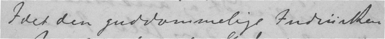Idet den guddommelige Indviklen
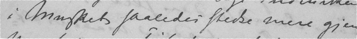i Msket saaledes stedse mere gjen-
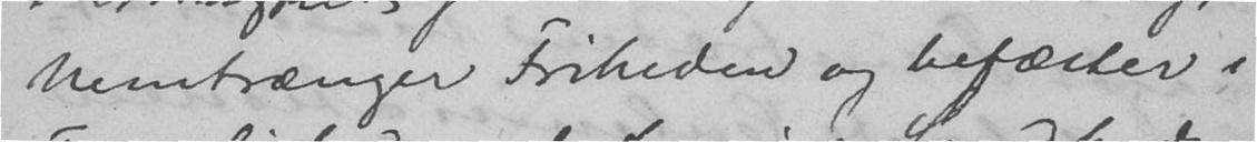nemtrænger Friheden og befæster i
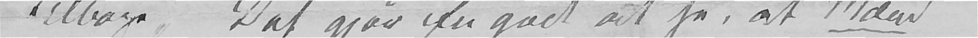tilbage. Det gjør En godt at se, at Mænd
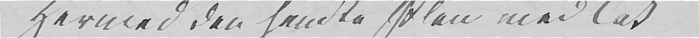Hermed den sendte Plan med Tak
